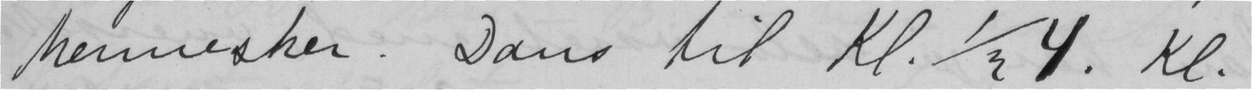Mennesker. Dans til Kl. ½ 4. Kl.
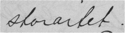storartet.
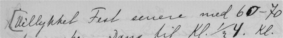Vellykket Fest senere med 60-70
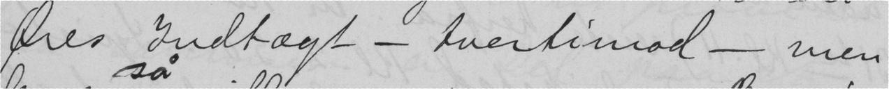Øres Indtægt - tvertimod - men
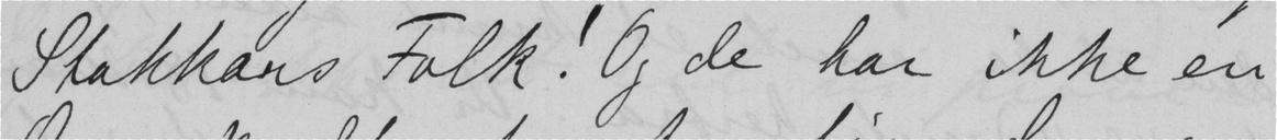Stakkars Folk! Og de har ikke en
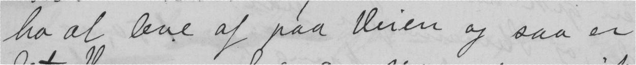ha at leve af paa veien og saa er
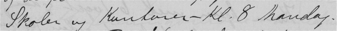Skoler og Kontorer - Kl. 8 Mandag.
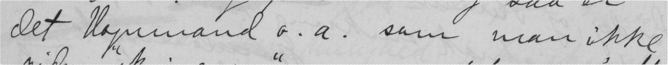det Vognmand o. a. som man ikke
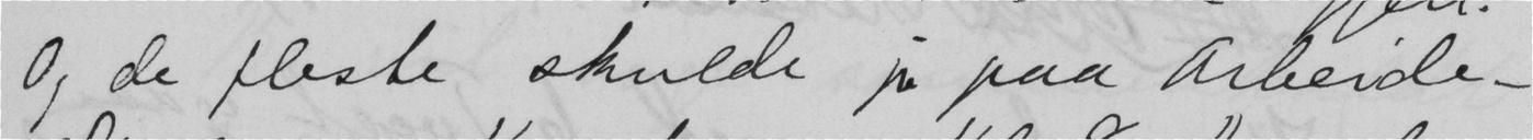Og de fleste skulde jo paa arveide -
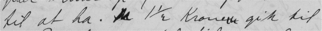til at ha.  1 1/2 Kroner gik til
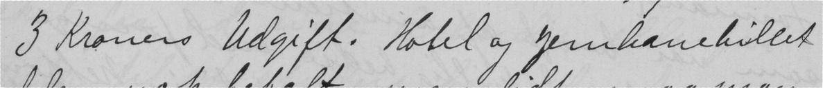3 Kroners Udgift. Hotel og jerbanebillet
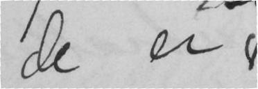de er
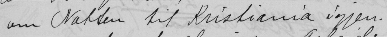om Natten til Kristiania igjen.
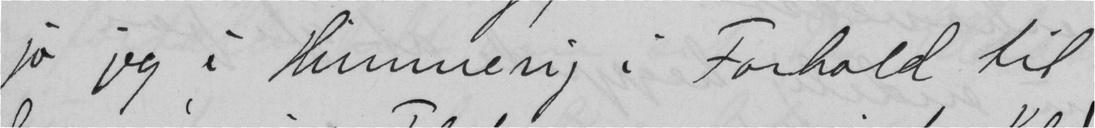jo jeg i Himmerig i Forhold til
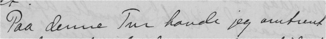Paa denne Tur havde jeg omtrent
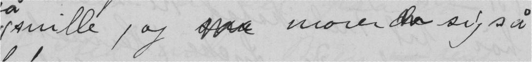snille, og  morer  sig så
